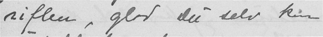riflen, glad du selv kom
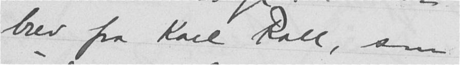brev fra Karl Roll, som
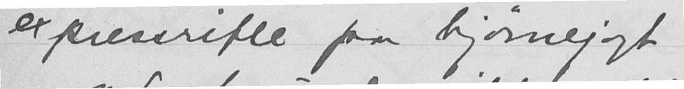expressrifle paa bjørnejagt
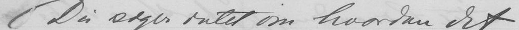Du siger intet om hvordan det
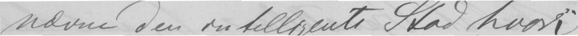nævne den intelligente Stad hvori
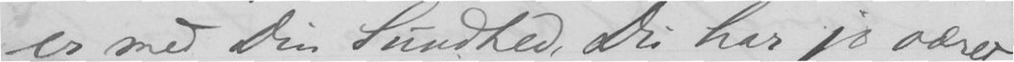er med Din Sundhed, Du har jo været
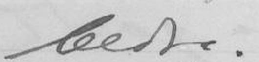bedre.
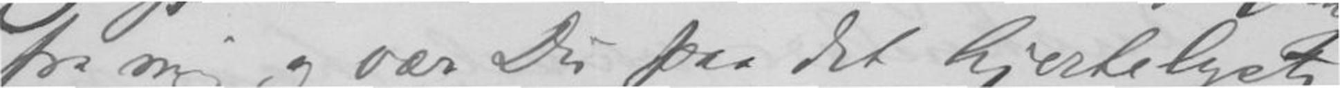fra mig og vær Du paa det hjerteligste
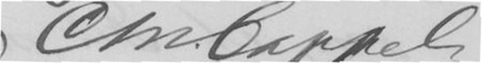Chr. Cappelen
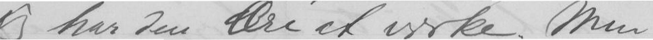jeg har den Ære at virke. Men
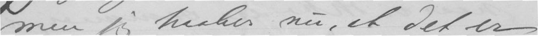men jeg haaber nu, at det er
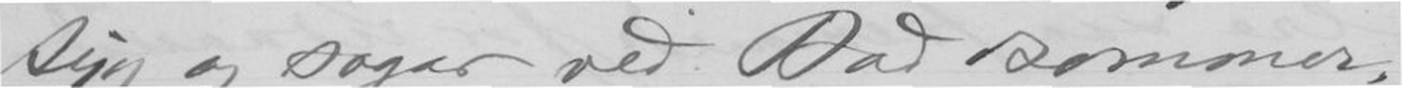syg og sogar ved Bad isommer,
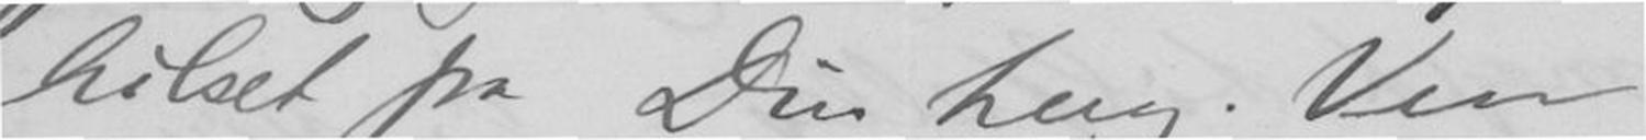hilset fra Din heng. Ven
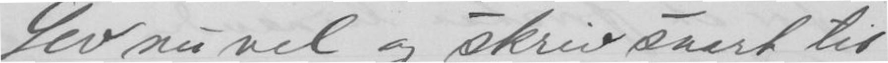Lev nu vel og skriv snart til
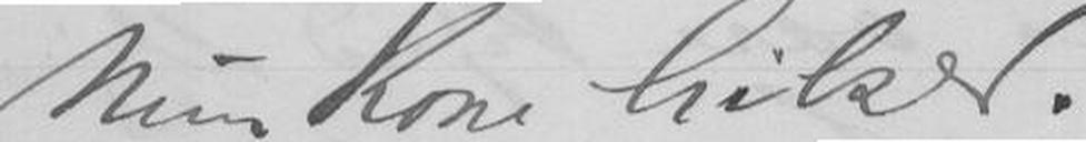Min Kone hilser
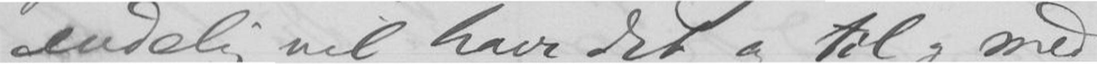endelig vil have det og til og med
